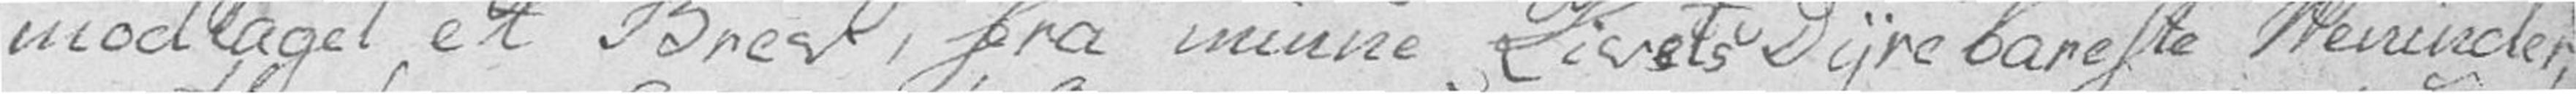modtaget et Brev, fra minne Livets Dyrebareste Veninder,
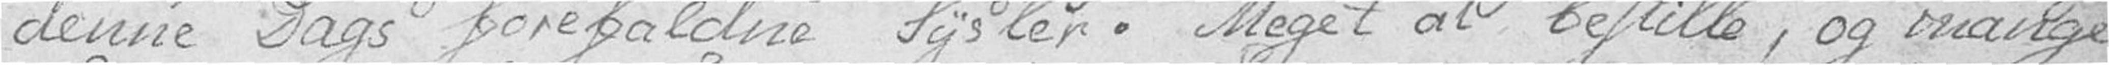denne Dags forefaldne Sysler. Meget at bestille, og mange
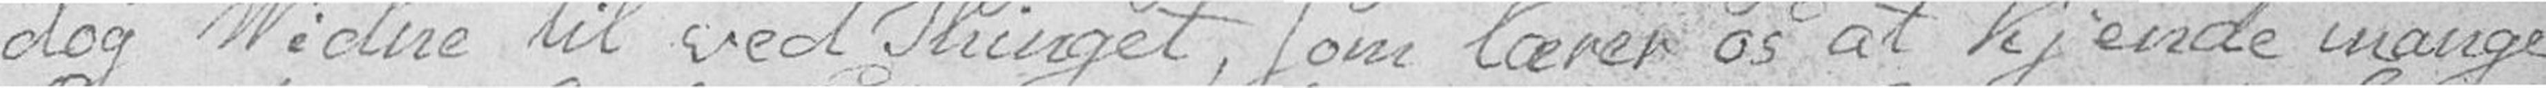dog Vidne til ved Thinget, som lærer os at kjende mange
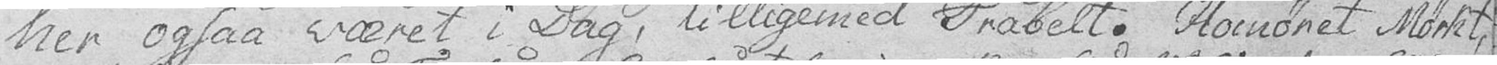her ogsaa været i Dag, tilligemed Trabelt. Homøret Mørkt,
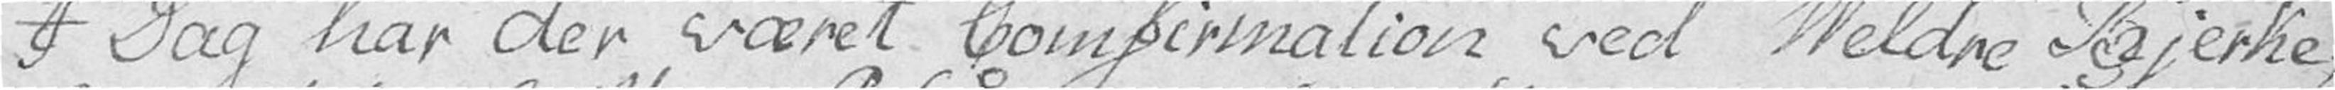I Dag har der været Comfirmation ved Veldre Kjerke,
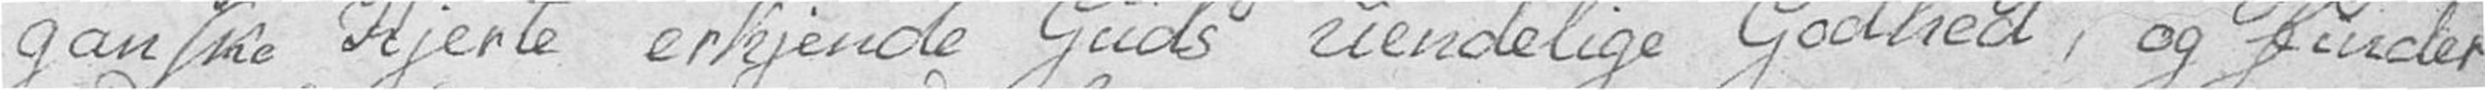ganske Hjerte erkjende Guds uendelige Godhed, og finder
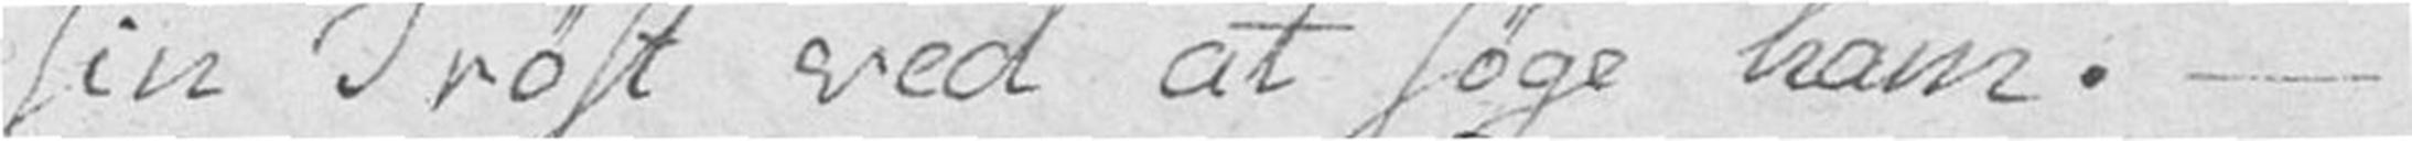sin Trøst ved at søge ham. –
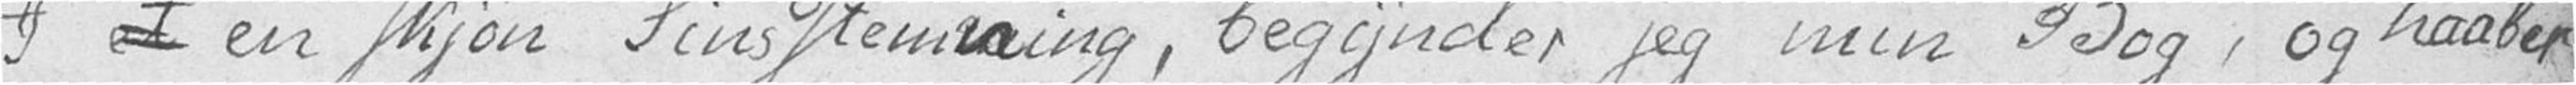I et en skjøn Sinsstemning, begynder jeg min Bog, og haaber
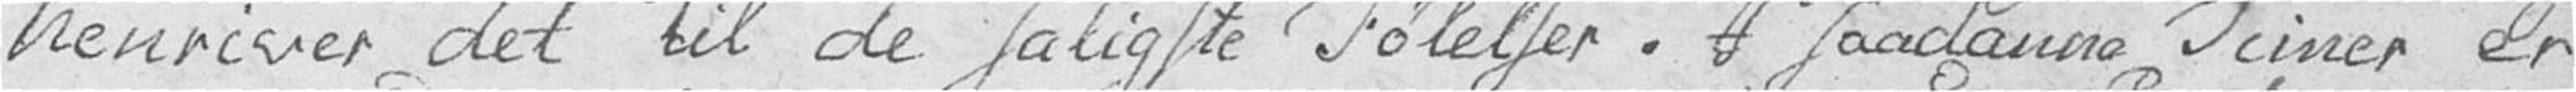henriver det til de saligste Følelser. I saadanne Timer er
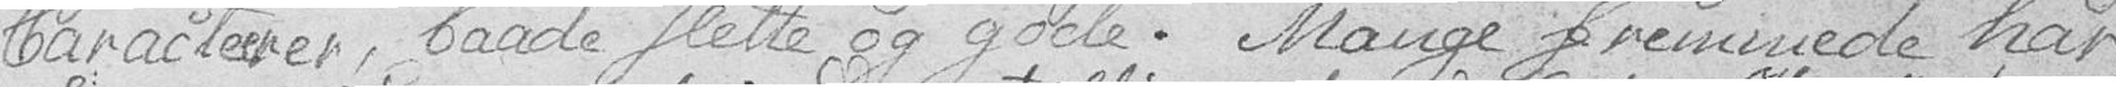Caracterer, baade slette og gode. Mange fremmede har
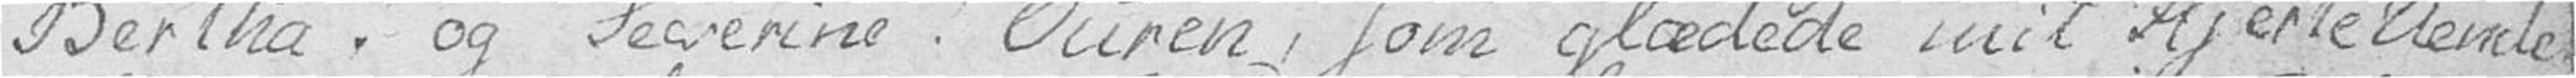Bertha! og Severine! Ouren, som glædede mit Hjerte Uende
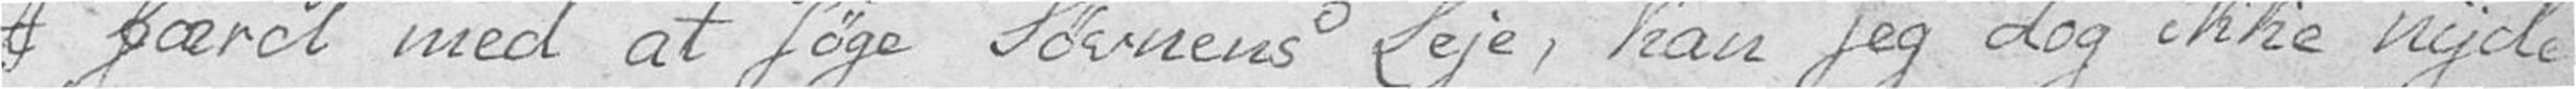I færd med at søge Søvnens Leje, kan jeg dog ikke nyde
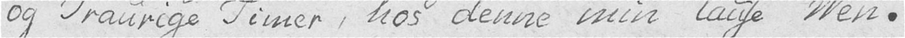og Traurige Timer, hos denne min tause Ven.
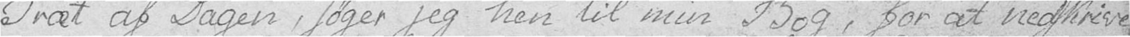Træt af Dagen, søger jeg hen til min Bog, for at nedskrive
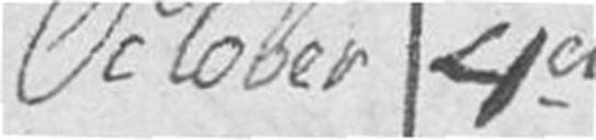October 4d
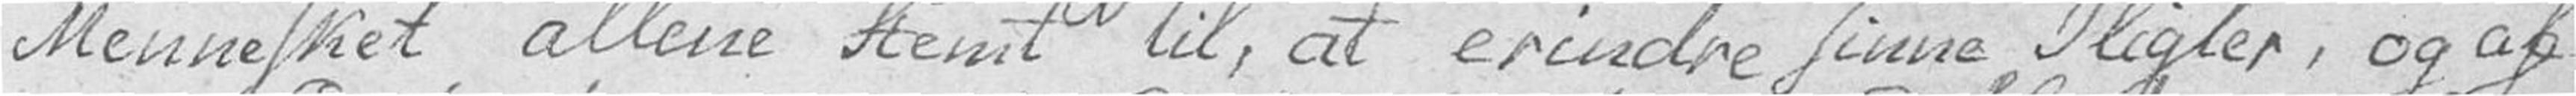Mennesket allene Stemt til, at erindre sinne Pligter, og af
